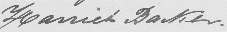Harriet Backer.
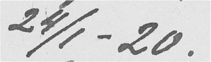24/1 - 20.
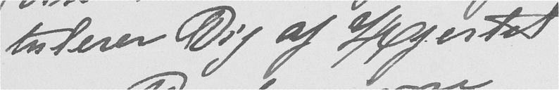tulerer Dig af Hjertet
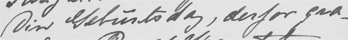Din Geburtsdag, derfor gra-
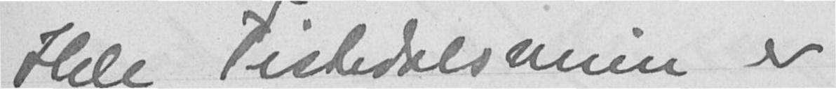Hele Tistedalsveien er
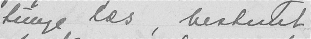tunge læs, bestemt
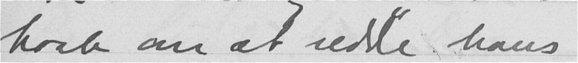haab om at redde hans
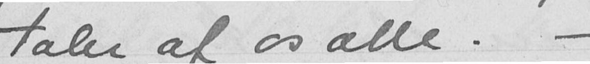taler af os alle. -
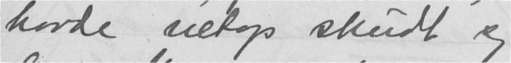havde netop skudt sig
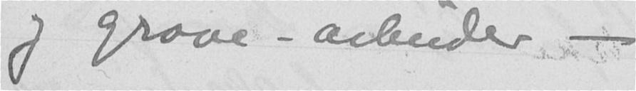og grave-arbeider -
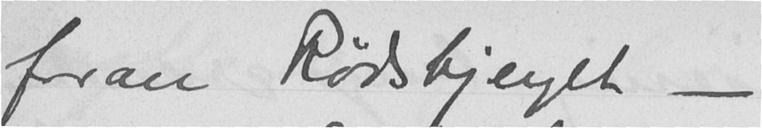foran Rødsbjerget -
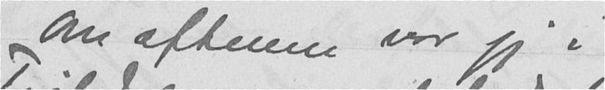Om aftenen var jeg i
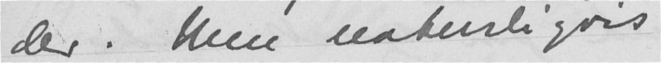der. Men naturligvis
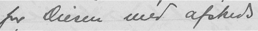for Diesen med afskeds
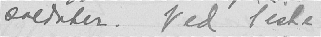soldater. Ved Tiste
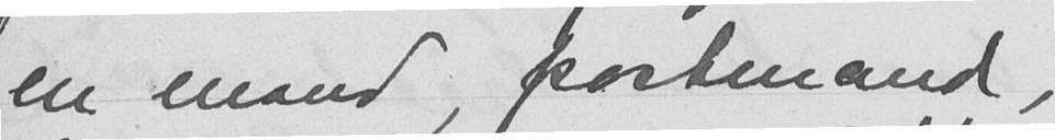en mand, postmand,
